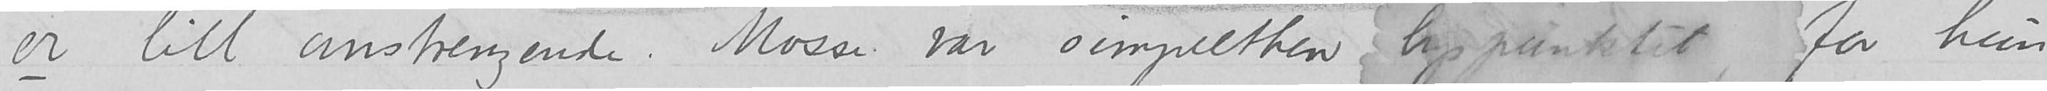er litt anstrengende.  Mosse var simpelthen lyspunktet, for hun
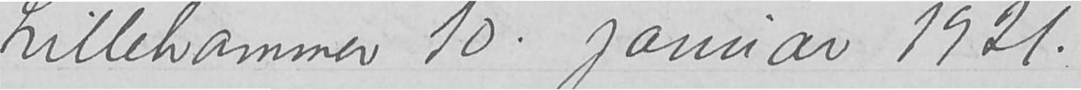Lillehammer 10. januar 1921.
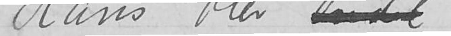Hans blev endel svært
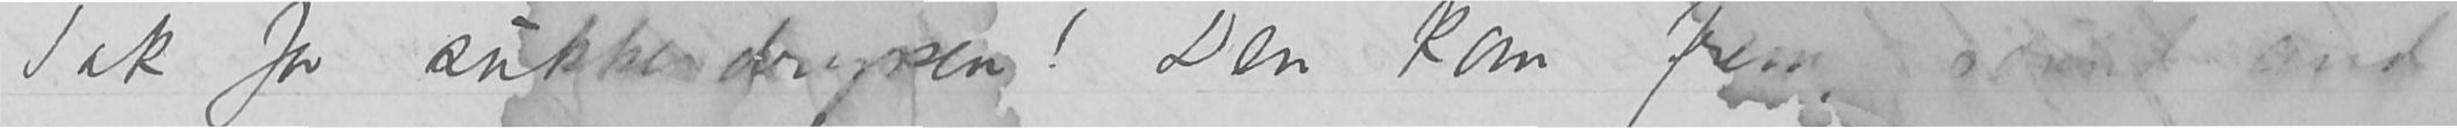Tak for sukkerdryssen!  Den kom frem sound and safe –
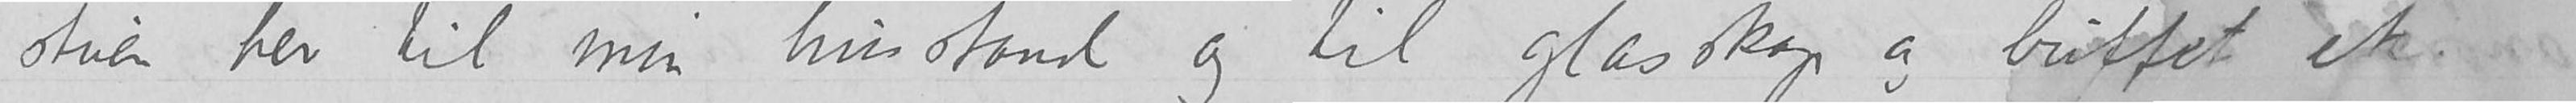stuen her til min husstand og til glasskap og buffet etc.
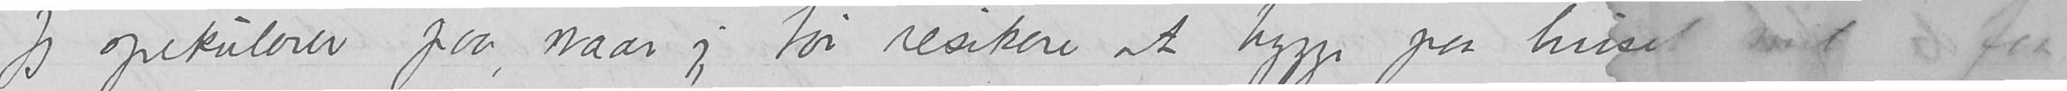Jeg spekulerer paa, naar jeg tør reisikere at bygge paa huset mit
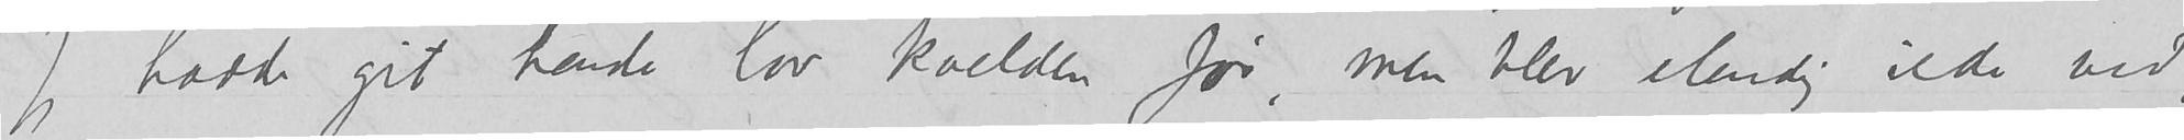Jeg hadde git hende lov kvelden før, men blev elendig ilde ved,
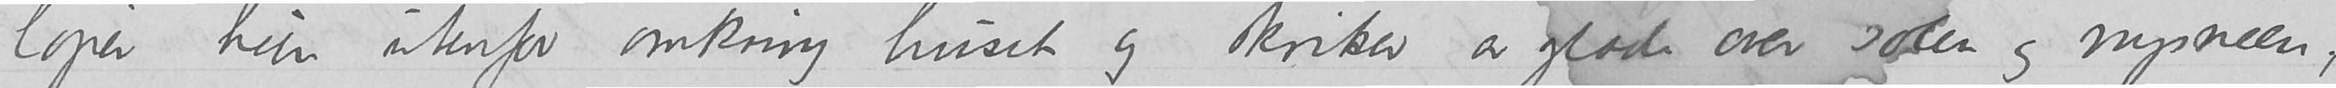løper hun utenfor omkring huset og skriker av glæde over solen og nysneen,
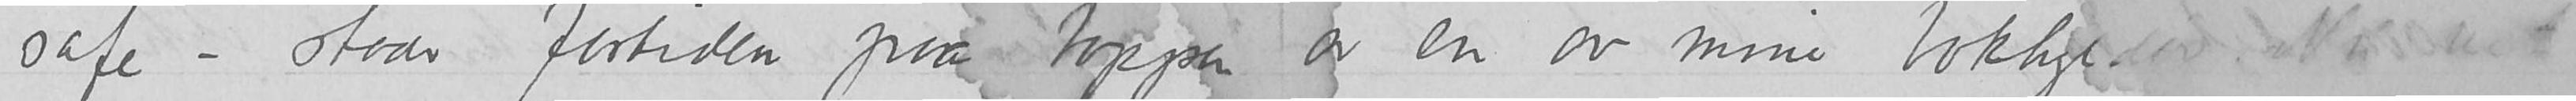staar fortiden paa toppen av en av mine bokhyller. Nu vet
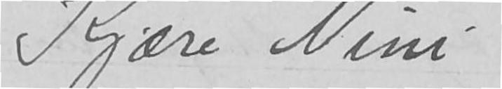Kjære Nini
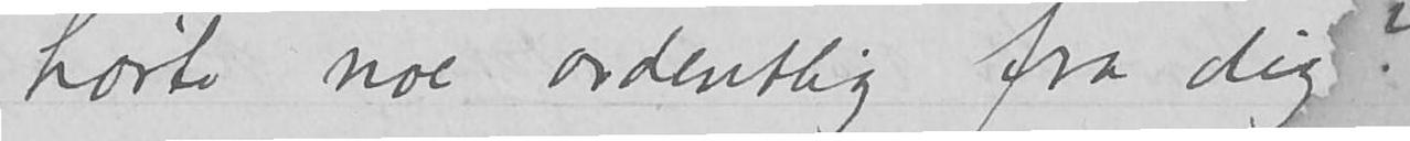hørte noe ordentlig fra dig?
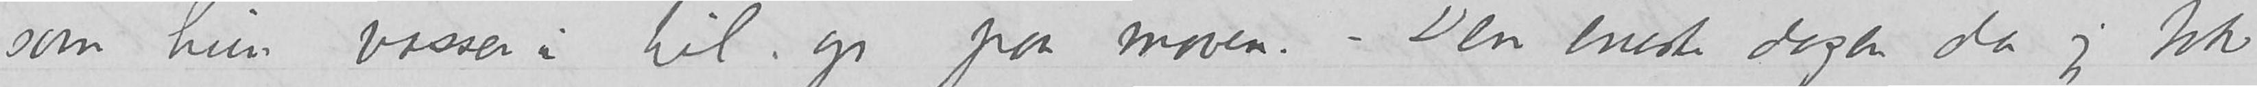som hun vasser i til op paa maven. –Den eneste dagen da jeg fik
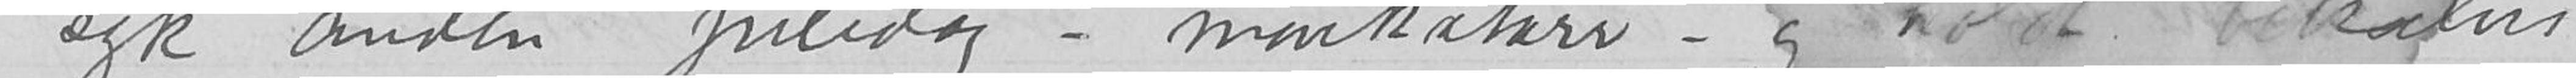syk anden juledag – mavekatarr – og holdt vekselvis
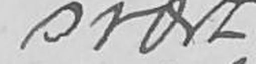svært
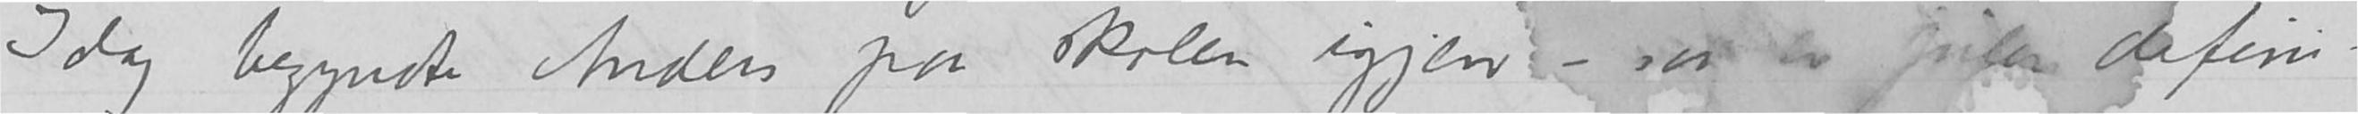I dag begyndteer Anders paa skolen igjen – saa er julen defini
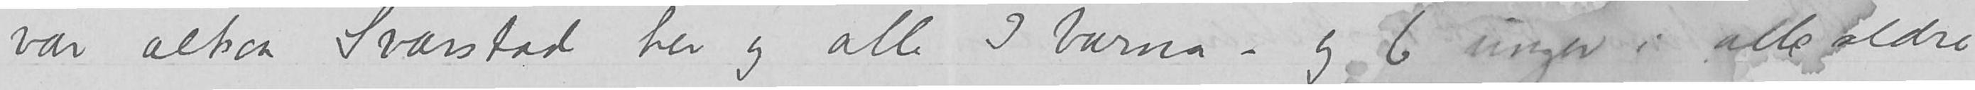var altsaa Svarstad her og alle 3 barna – og 6 unger i alle aldre
